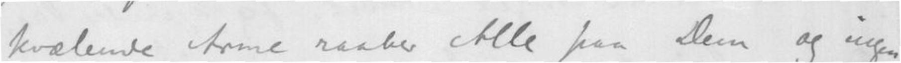kvælende Arme raades Alle paa Dem og ingen
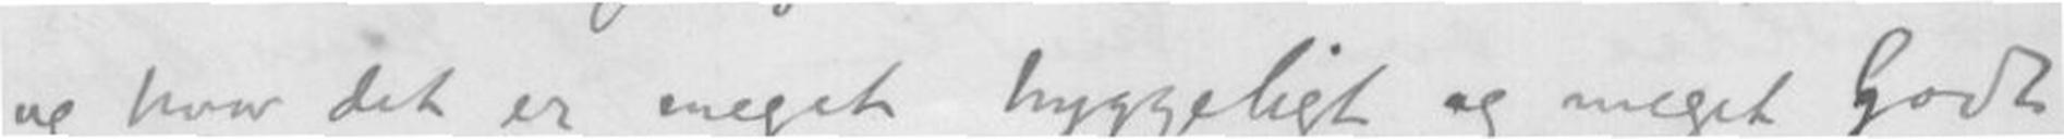op hvor det er meget hyggeligt og meget Godt
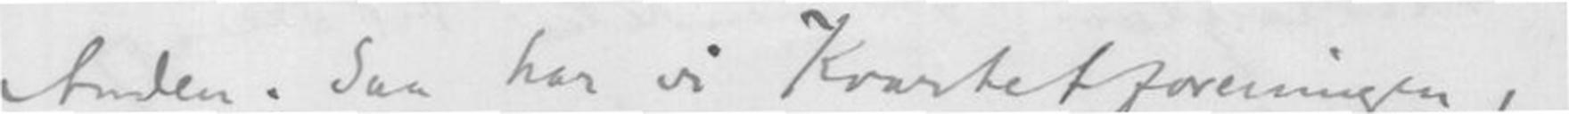Anden. Saa har vi Kvartetforeningen,
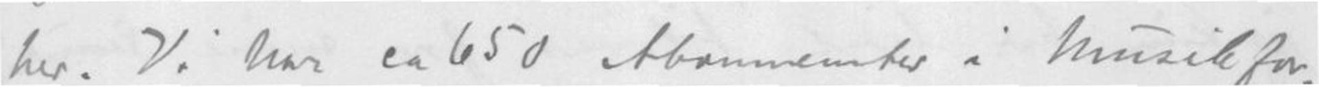her. Vi har ca.650 Abonnenter i Musikfor-
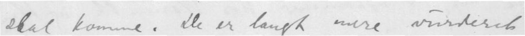skal komme. De er langt mere vurderet
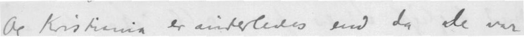Og Kristiania er anderledes end da de var
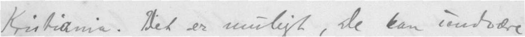Kristiania. Det er muligt, de kan undvære
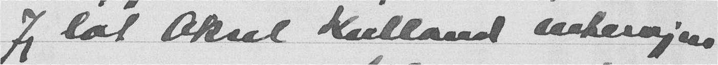Jeg lot Aksel Kielland intervjue
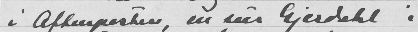i Aftenposten, en sur Gjerdahl i
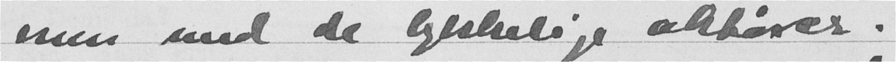men med de lykkelige aktører.
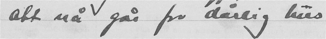alt nå går for dårlig hus. -
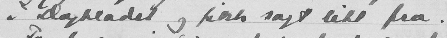i Dagbladet og fikk sagt litt fra.
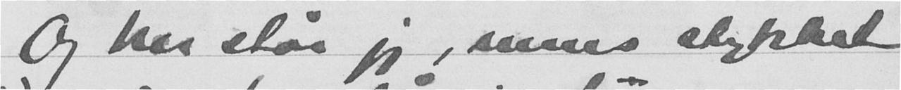Og her står jeg, mens stykket
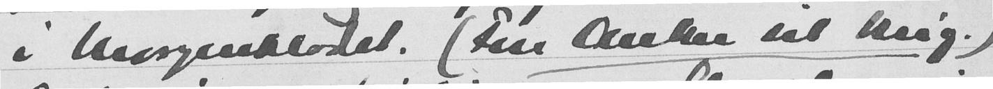i Morgenbladet. (Fru Anker til krig.)
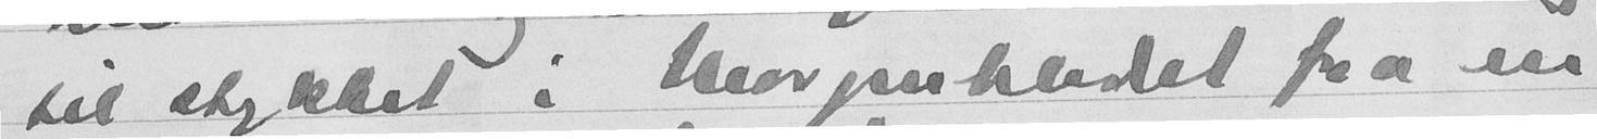til stykket i Morgenbladet fra en
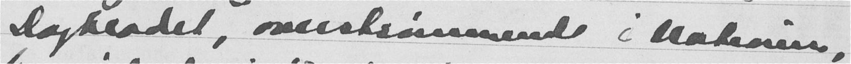Dagbladet, overstrømmende i Nationen,
